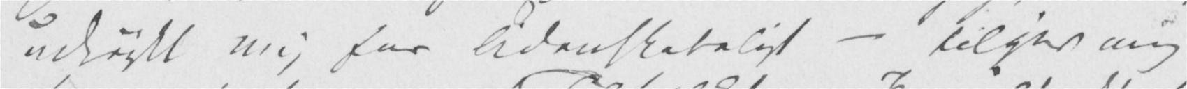udtrykt mig for lidenskabeligt – tilgiv mig
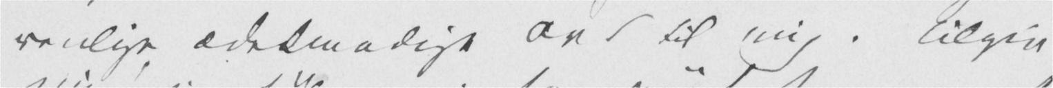venlige ædelmodige Ord til mig. Tilgiv
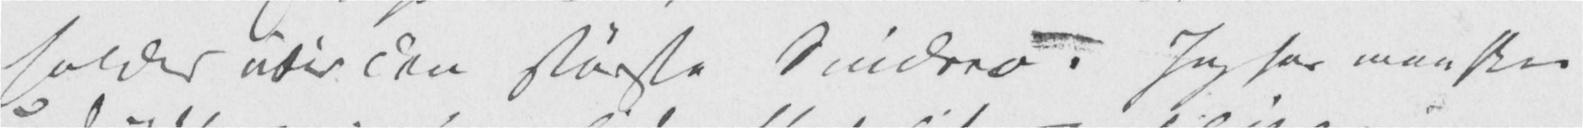holdes under den største Sindsro. Jeg har maaskee
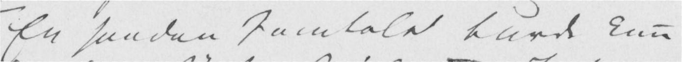En saadan Samtale burde kun
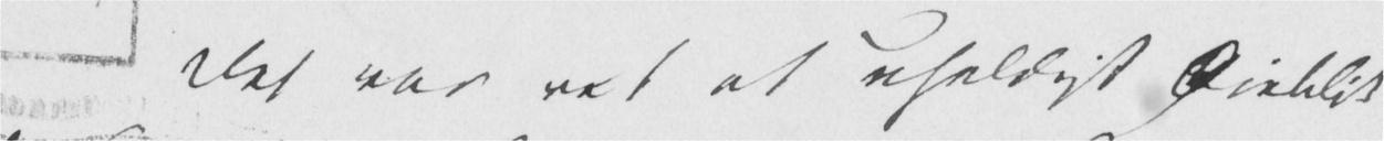Det var ret et uheldigt Øieblik
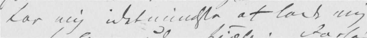Lov mig idetmindste at lade mig
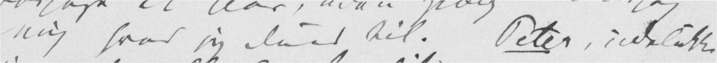mig hvad jeg duer til. Peter, udelukker
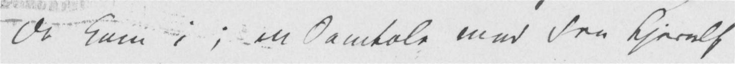De kom i; en Samtale med Fru Kjerulf
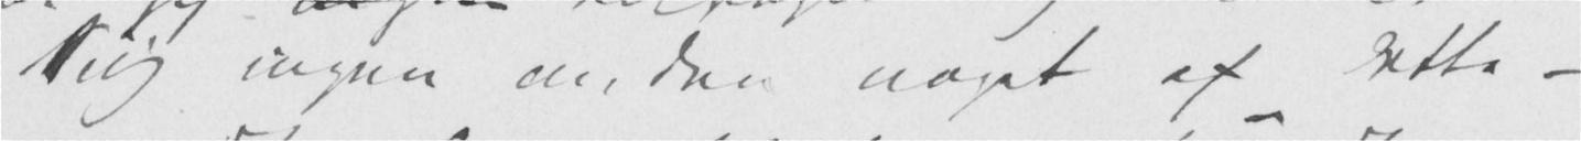Siig ingen anden noget af dette –
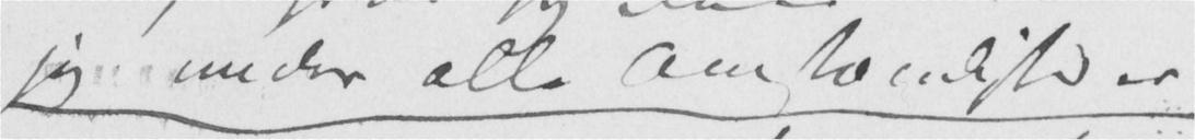jeg under alle Omstændigheder
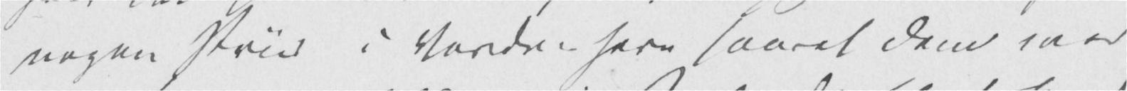nogen Priis i Verden have saaret Dem med
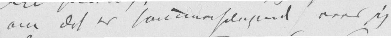om det er sammenhængende veed jeg
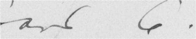Deres C.
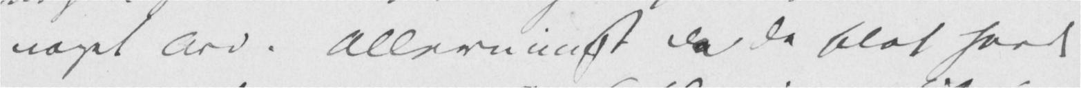noget Ord. Allermindst da De blot havde
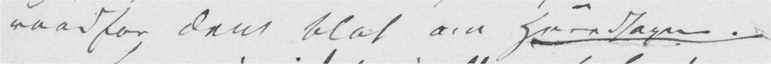raadfør Dem blot om Hovedsagen.
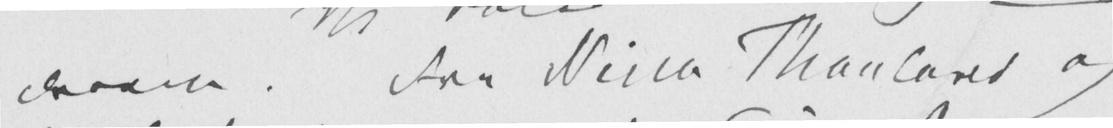derom. Fru Nina Thaulow og
